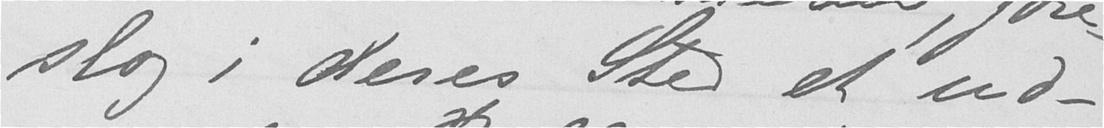slog i deres Sted et ud-
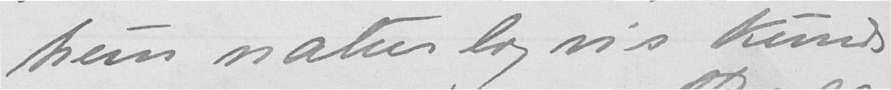hun naturligvis kunde
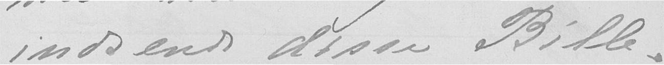indsende disse Bille.
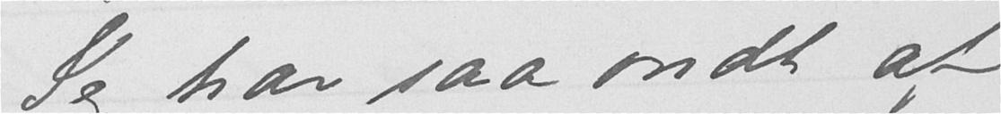Jeg har saa ondt af
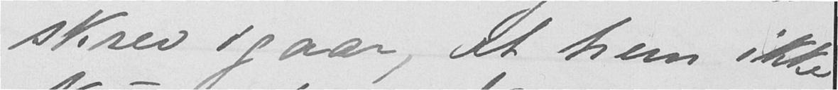skrev igaar, at hun ikke
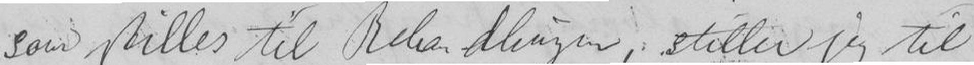som stilles til Behandlingen, stiller jeg til
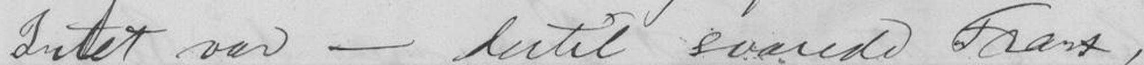Intet var - dertil svarede Franz,
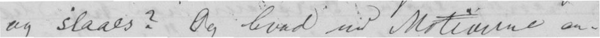og slaaes? Og hvad nu Motiverne an-
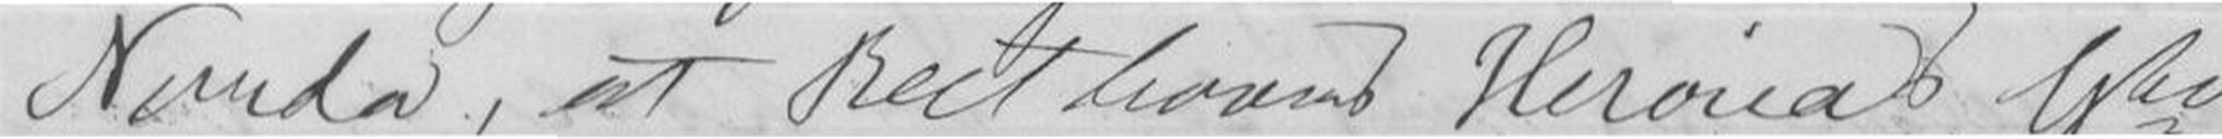Neruda, at Beethovens Heroicas 1she
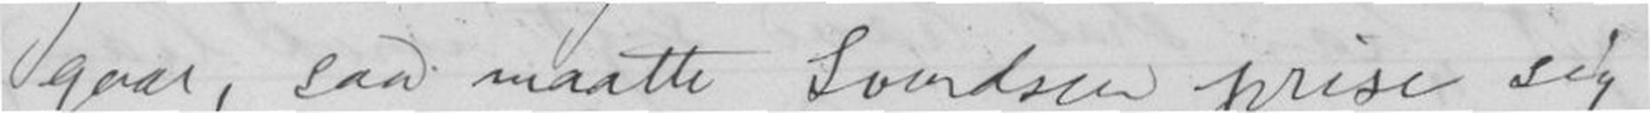gaar, saa maatte Svendsen prise sig
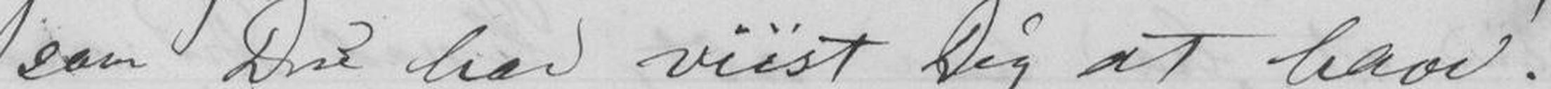som Du har viist Dig at have.
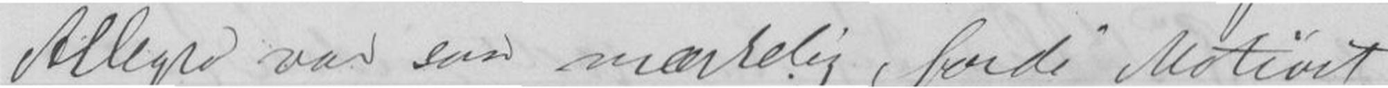Allegro var saa mærkelig, fordi Motivet
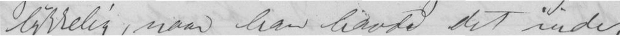lykkelig, naar han havde det inde,
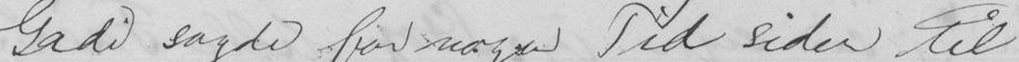Gade sagde for nogen Tid siden til
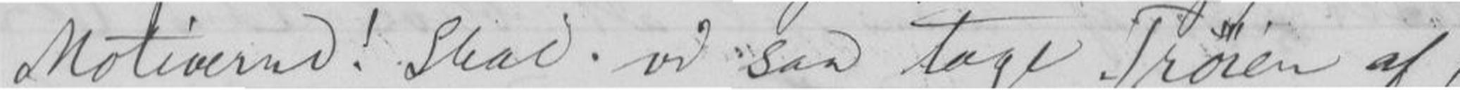Motiverne! Skal vi saa tage Trøien af,
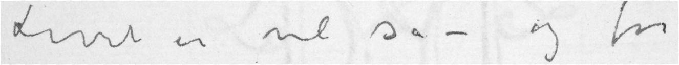Livet er vel så – og for
